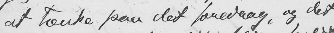at tænke paa det foredrag, og det
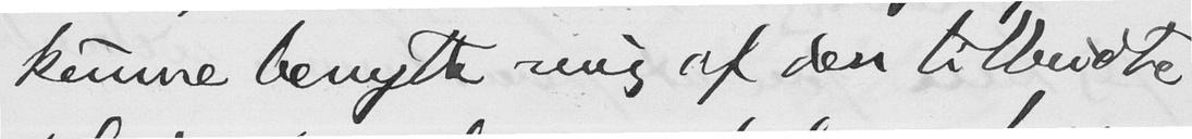kunne benytte mig af den tilbudte
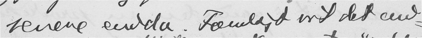senere endda. Fræmlagd vil det end-
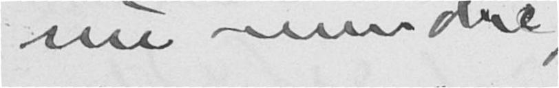nu mindre
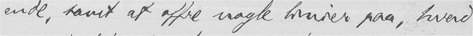ende, samt at offre nogle linier paa, hvad
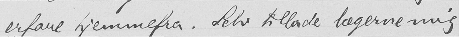erfare hjemmefra. Selv tillade lægerne mig
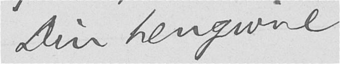Din hengivne
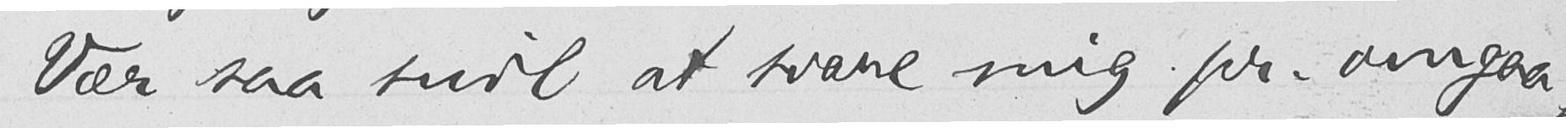Vær saa snil at svare mig pr. omgaa-
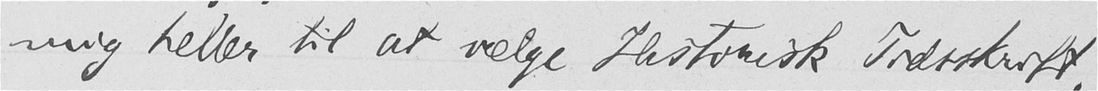mig heller til at vælge Historisk Tidsskrift,
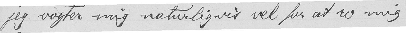jeg vogter mig naturligvis vel for at ro mig
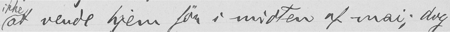at vende hjem før i midten af mai; dog
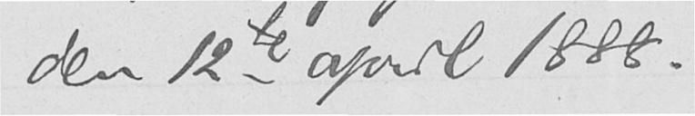den 12te april 1888.
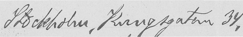Stockholm, Kungsgatan 34,
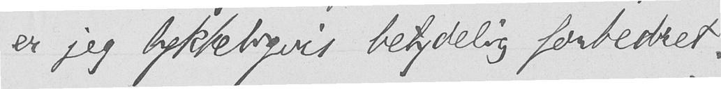er jeg lykkeligvis betydelig forbedret.
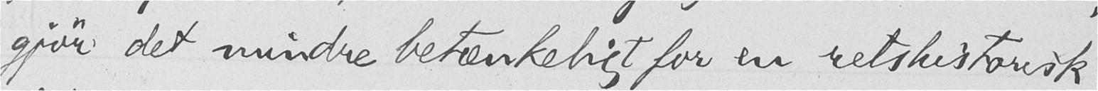gjør det mindre betænkeligt for en retshistorisk
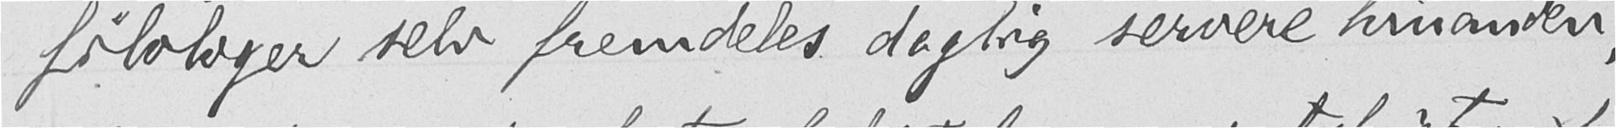filologer selv fremdeles daglig servere hinanden,
